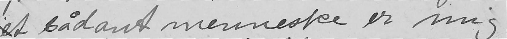et sådant menneske er mig
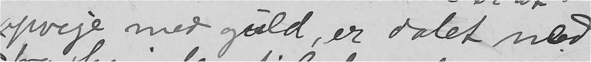opveje med guld, er dalet ned
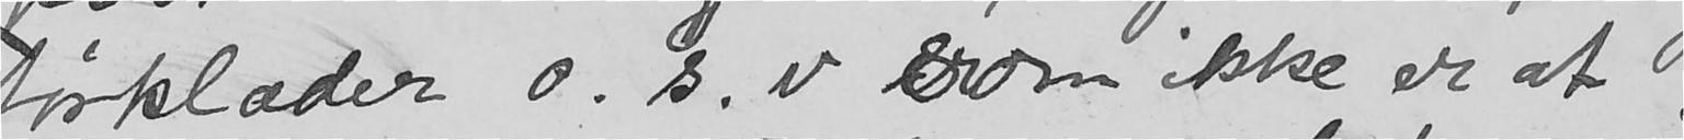tørklæder o.s.v som ikke er at
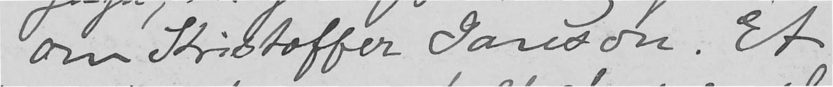om Kristoffer Janson. Et
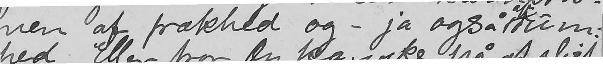nen af frækhed og - ja også dum-
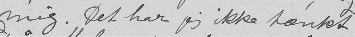mig. Det har jeg ikke tænkt
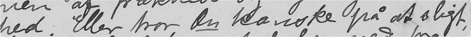hed. Eller tror du kanske på at sligt,
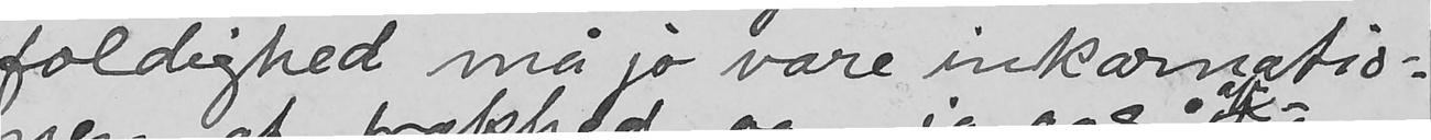foldighed må jo være inkarnatis-
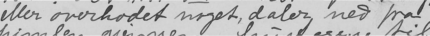eller overhodet noget, daler ned fra
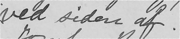ved siden af.
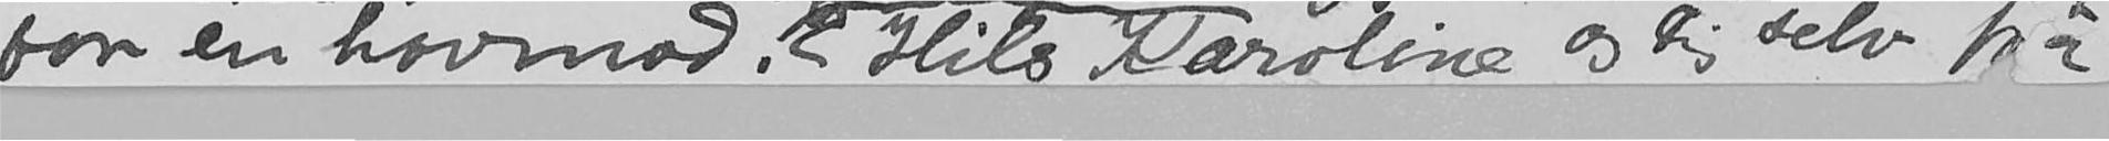for en hovmod? Hils Karoline og Dig selv fra
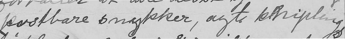kostbare snykker, ægte kniplings
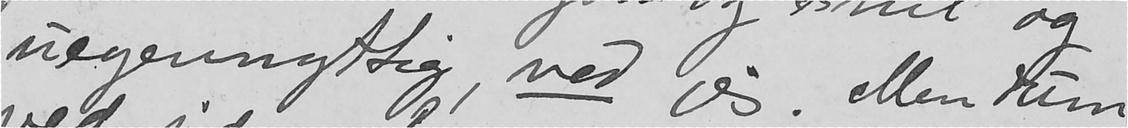uegennyttig, ved jeg. Men kun
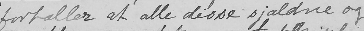fortæller at alle disse sjældne og
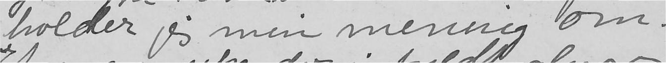holder jeg min mening om.
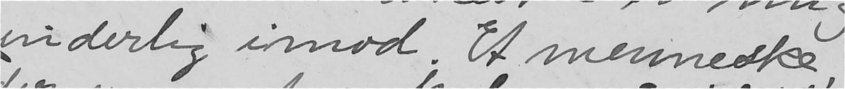inderlig imod. Et menneske,
